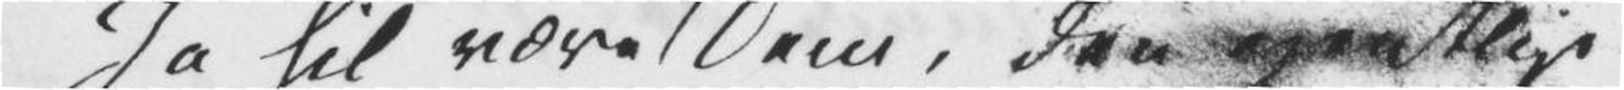Ja hil være Dem, den egentlige
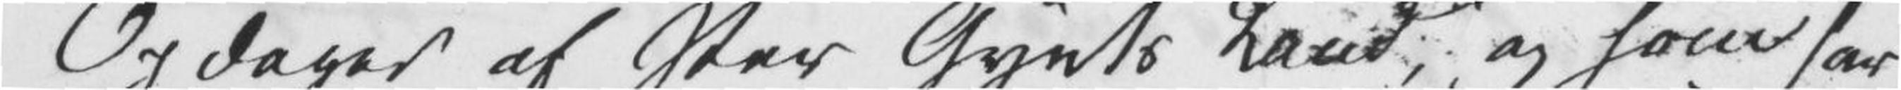Opdager af Per Gynts Land, og som har
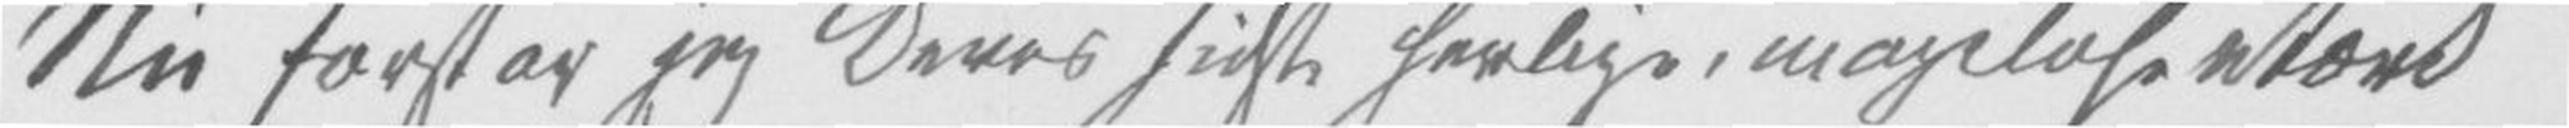Nu forstår jeg Deres sidste herlige, mageløse Værk
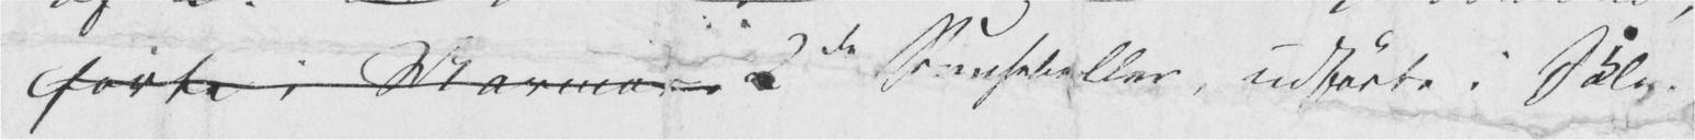førte i Marmor. 2de Punseboller, udførte i Sølv.
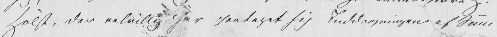Holst, der velvillig har paataget sig Inddragningen af Sum-
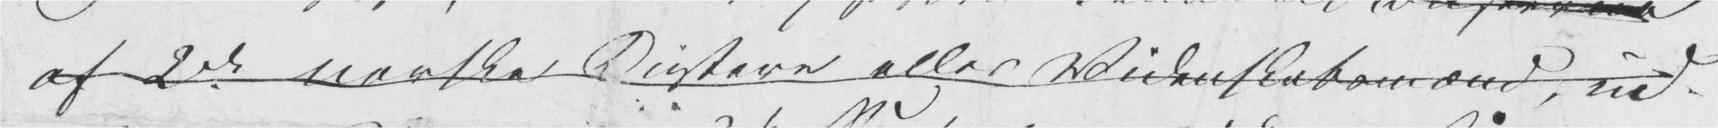af 2de norske Digtere eller Videnskabsmænd, ud-
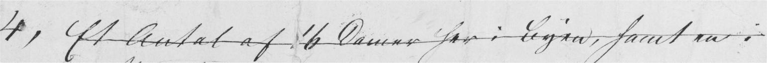4, Et Antal af 16 Damer her i Byen, samt en i
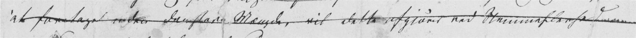at foretage inden den store Mængde, vil dette afgjøres ved Stemmeflerhed
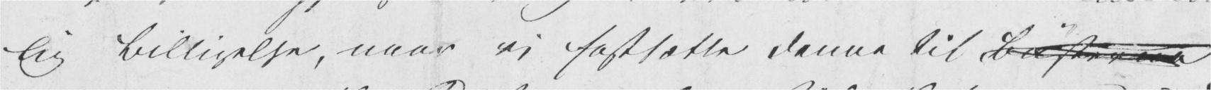lig Billigelse, naar vi fastsætte denne til Buster
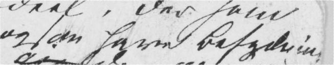ogsaa have Betydning
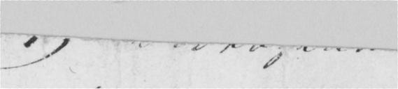<...>
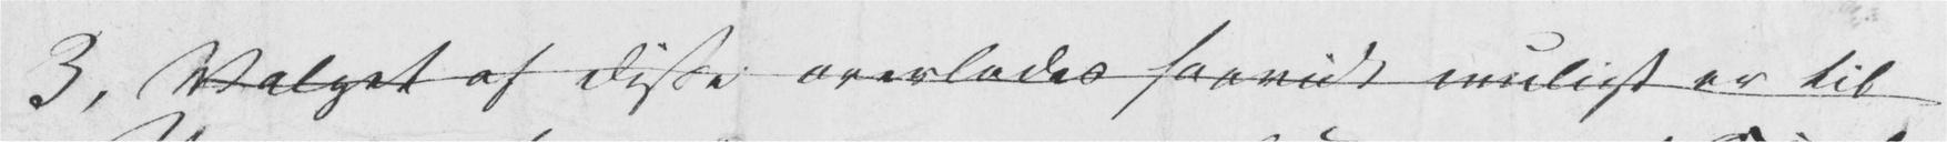3, Valget af disse overlades saavidt muligt er til
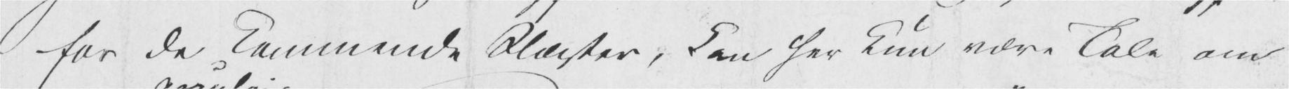for de kommende Slægter, kan her kun være Tale om
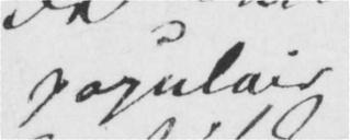populair
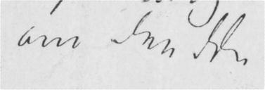<...>
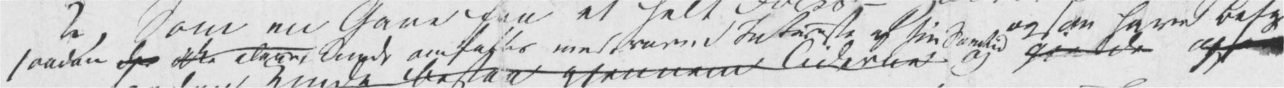saadan der ikke alene kunde omfattes med varm Interesse af sin Samtid gjelde ogsaa
